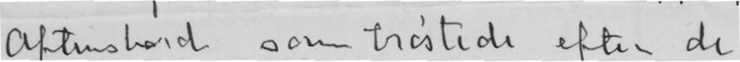Aftensbord som trøstede efter de
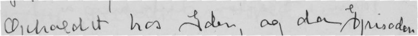Opholdet hos Eder, og da Episoden
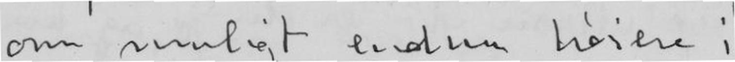om muligt endnu høiere i
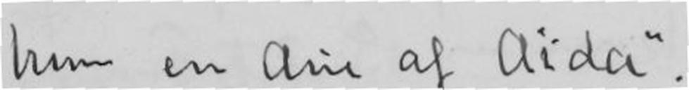hun en Arie af "Aida".
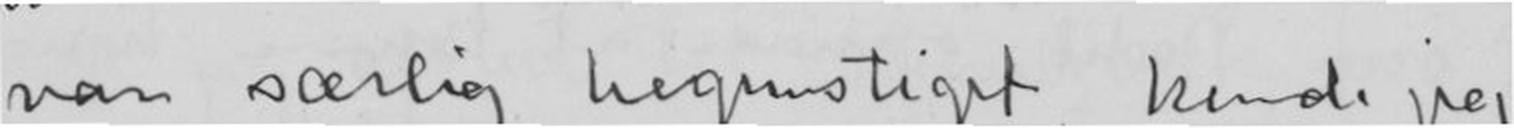var særlig begunstiget, kunde jeg
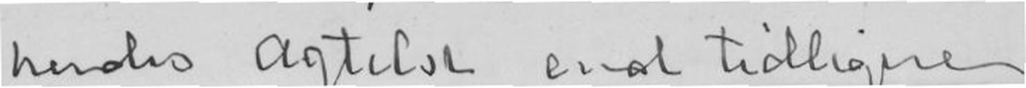hendes Agtelse end tidligere.
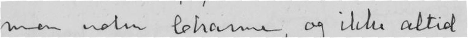men uden Charme, og ikke altid
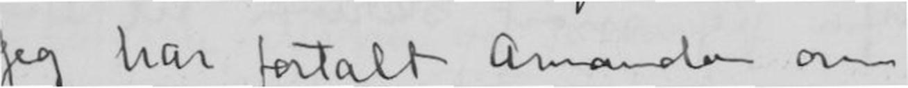Jeg har fortalt Amanda om
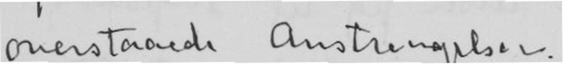overstaaende Anstrengelser.
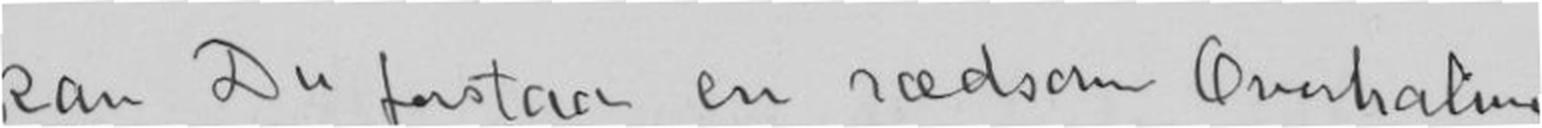kan Du forstaa en rædsom Overhaling.
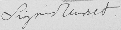Sigrid Undset
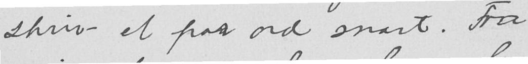skriv et par ord snart. Fra
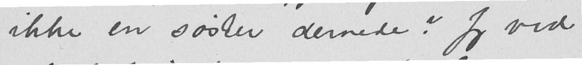ikke en søster dernede? Jeg ved
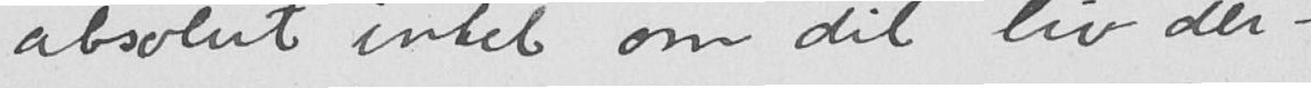absolut intet om dit liv der-
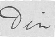Din
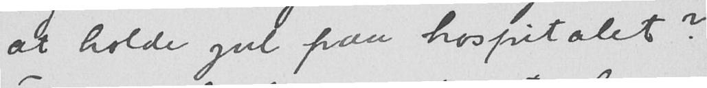at holde jul paa hospitalet?
